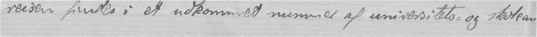reisen findes i et udkommet nummer af universitets- og skolean-
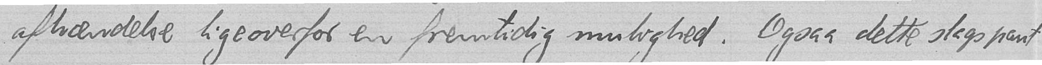afhændelse ligeovefor en fremtidig mulighed. Ogsaa dette slags pant
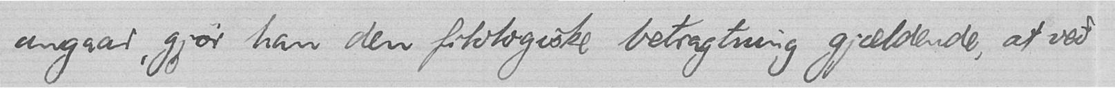angaar, gjør han den filologiske betragtning gjældende, at veð
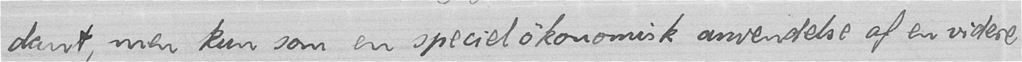dant, men kun som en speciel økonomisk anvendelse af en videre
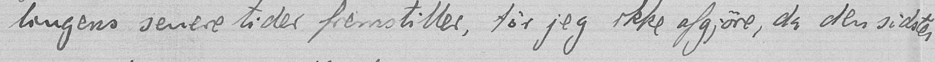lingens senere tider fremstiller, tør jeg ikke afgjøre, da den sidstes
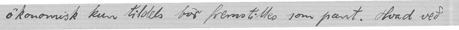økonomisk kun tildels bør fremstilles som pant. Hvad veð
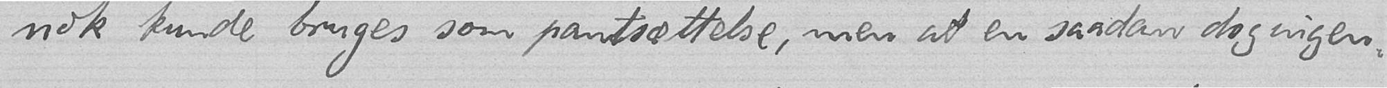nok kunde bruges som pantsættelse, men at en saadan dog ingen-
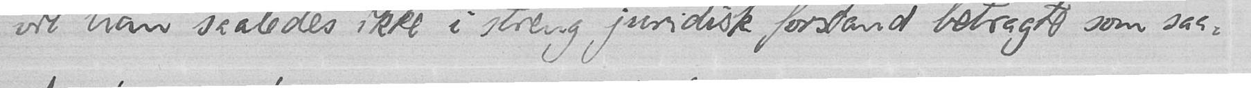vil han saaledes ikke i streng juridisk forstand betragte som saa-
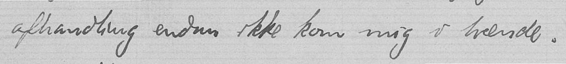afhandling endnu ikke kom mig i hænde.
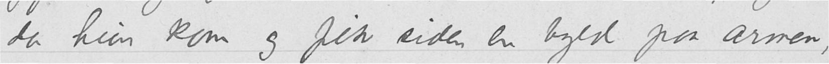da hun kom og fik siden en byld paa armen,
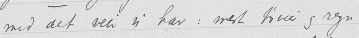med det veir vi har: mest tøveir og regn,
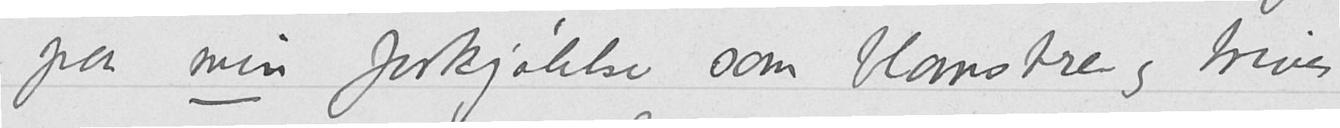paa min forkjølelse som blomstrer og trives
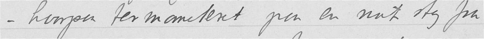- hvorpaa termometeret paa en nat steg fra
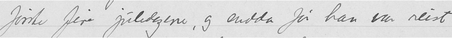første fire juledagene, og endda før han var reist
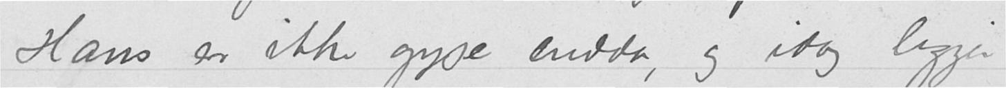- Hans er ikke oppe endda, og idag ligger
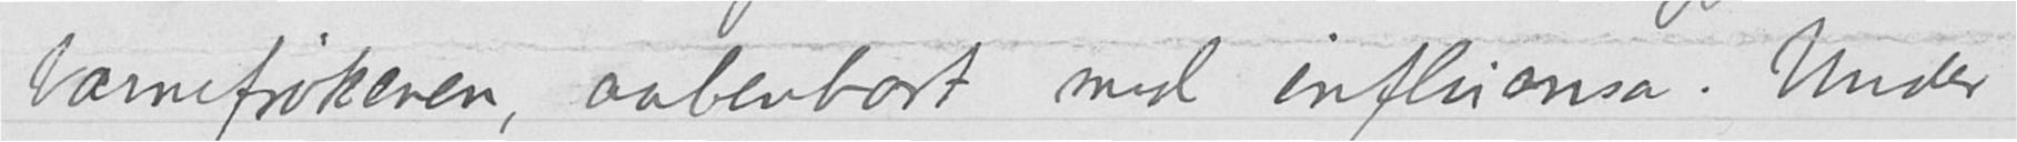barnefrøkenen, aabenbart med influensa. Under
